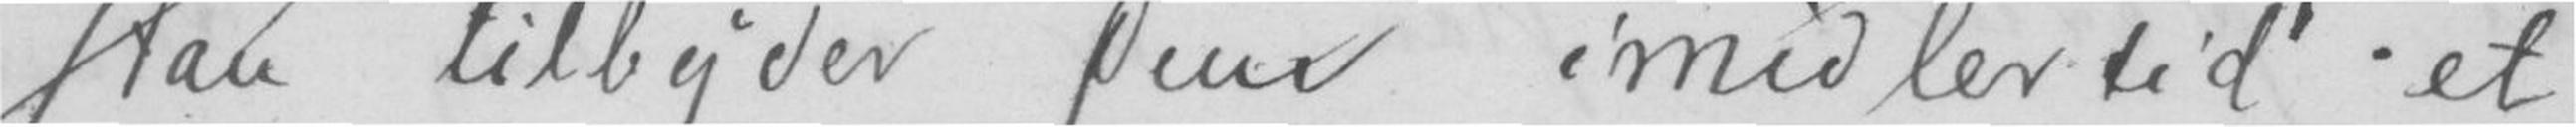Han tilbyder Deres imidlertid et
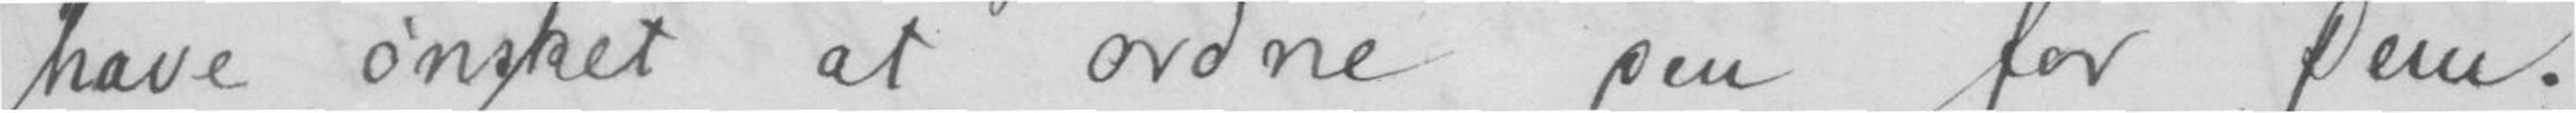have ønsket at ordne den for Dem.
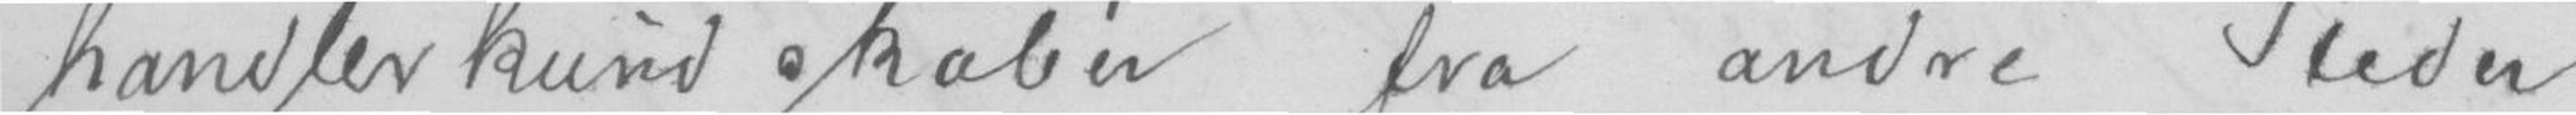handlerkundskaber fra andre Siden
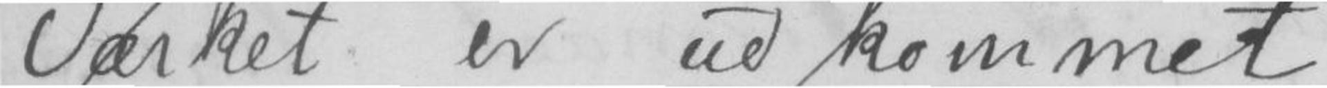Værket er udkommet.
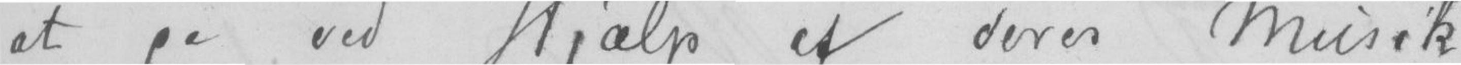at de ved Hjælp af deres Musik
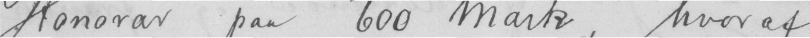Honorar paa 600 Mark, hvor af
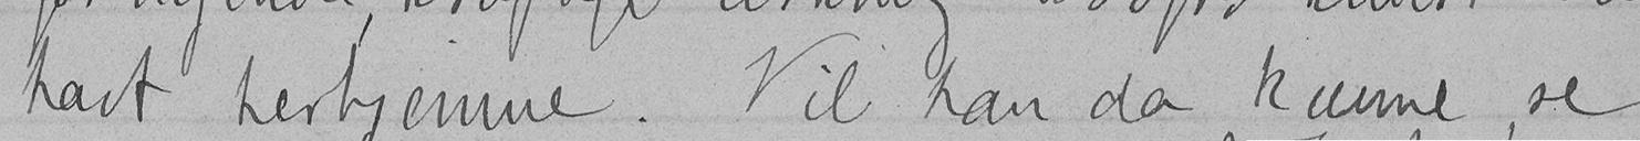havt herhjemme. Vil han da kunne se
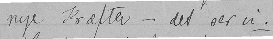nye Kræfter - det ser vi.
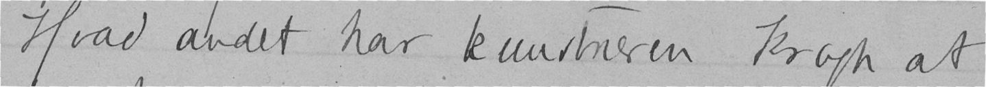Hvad andet har kunstneren Krogh at
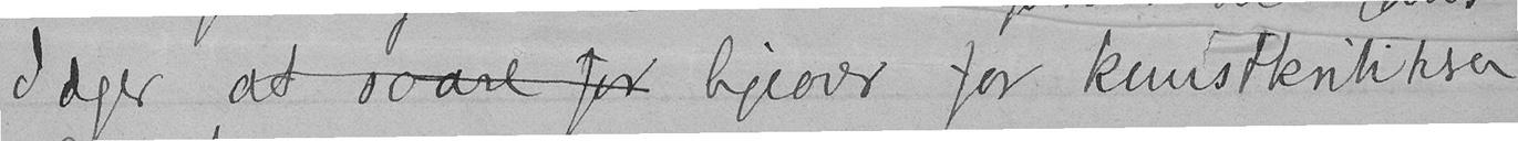Jæger at svare for ligeover for kunstkritikeren
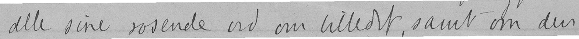alle sine rosende ord om billedet, samt om den
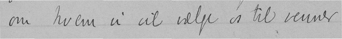om hvem vi vil vælge os til venner
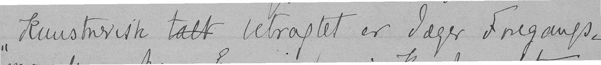"Kunstnerisk talt betragtet er Jæger Foregangs-
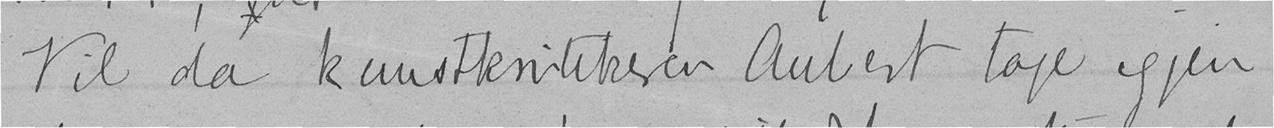Vil da kunstkritikeren Aubert tage igjen
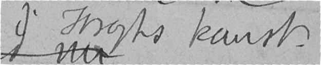i Kroghs kunst.
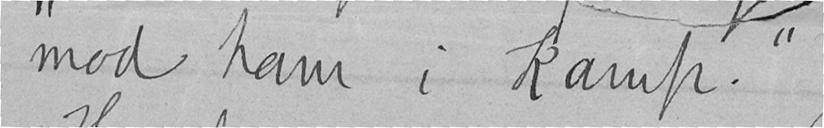mod ham i Kamp."
